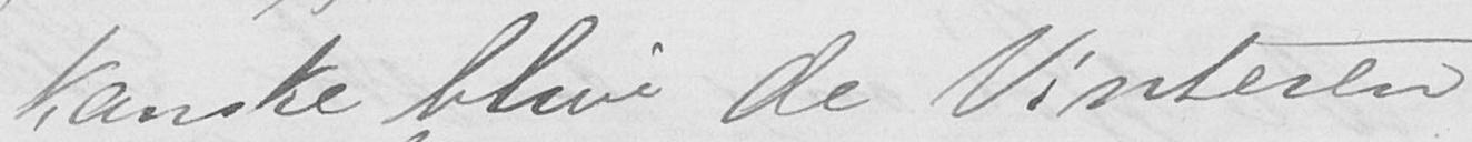kanske blive de Vinteren
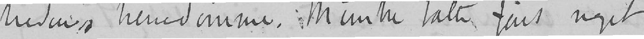hedens herredømme. Munthe talte først noget
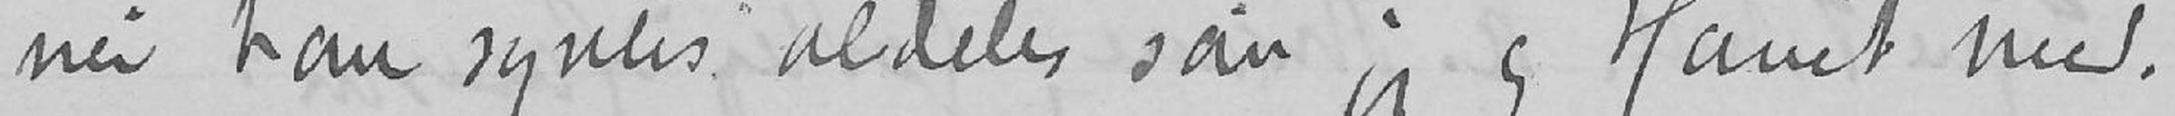nei han syntes aldeles som jeg og Harriet med.
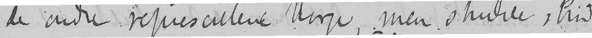de andre representere Norge, men skulde skriv-
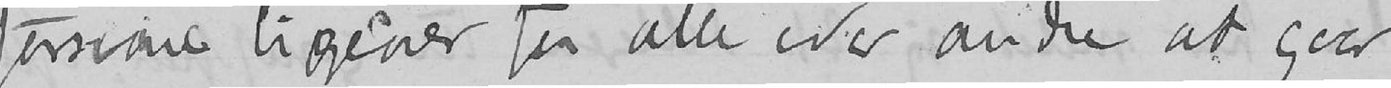forsvare ligeover for alle eder andre at gaa
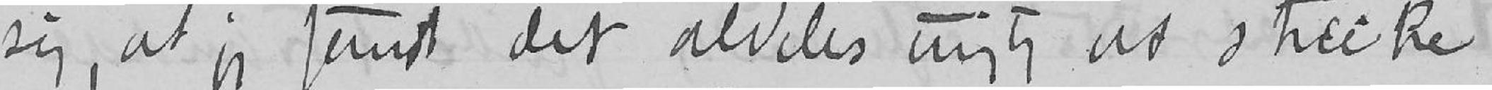sige, at jeg fandt det aldeles urigtig at streike
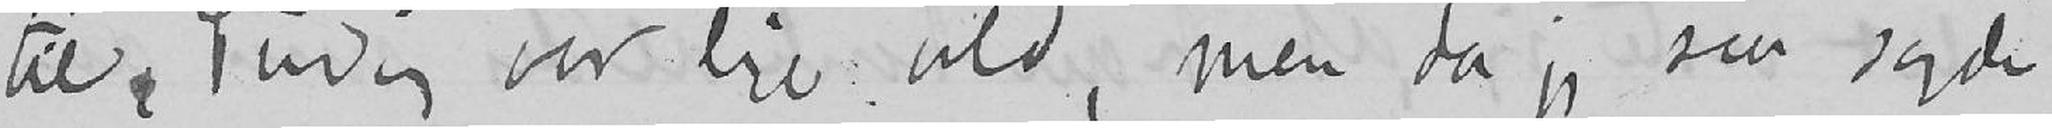til, Sinding var lige vild, men da jeg saa sagde,
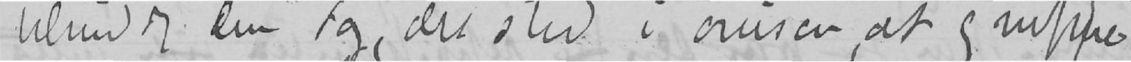til middag den dag, det stod i avisen, at gruppe
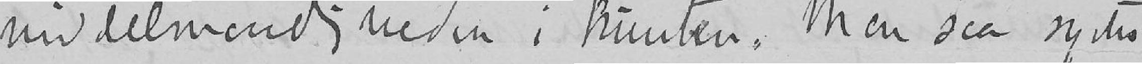middelmaadigheden i kunsten. Men saa syntes
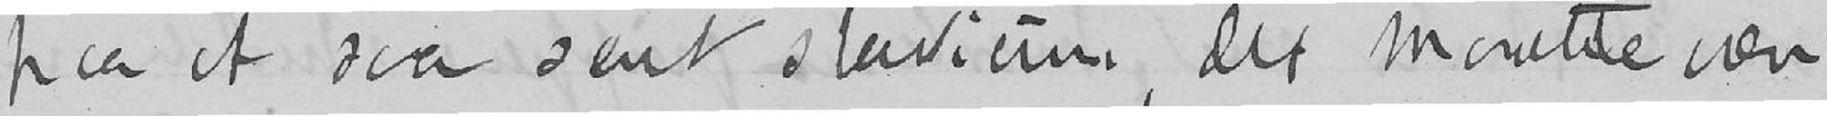paa et saa sent stadium, det maatte være
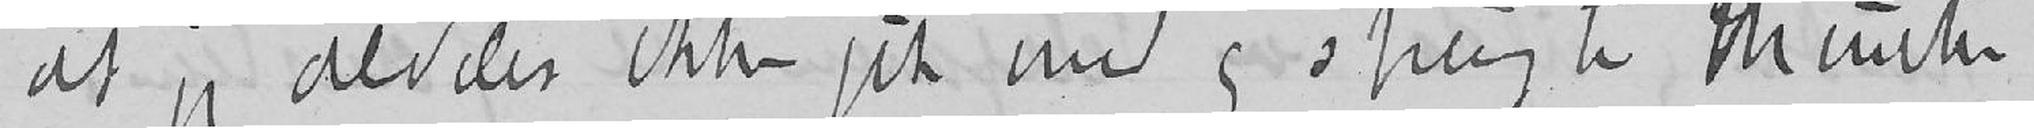at jeg aldeles ikke gik med og spurgte Munthe
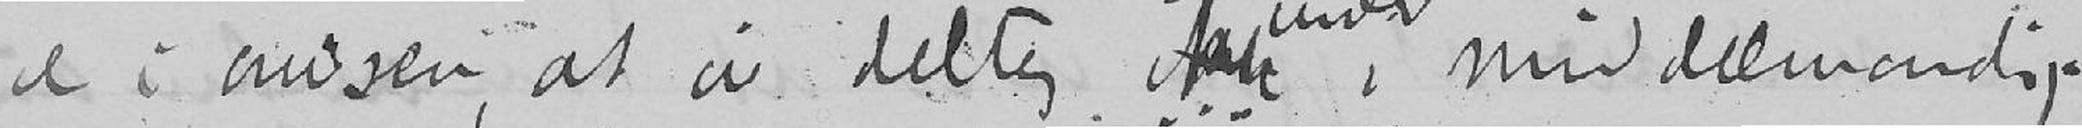e i avisen, at vi deltog ikke under middelmaadig-
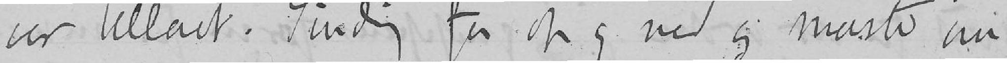var tilladt. Sinding for op og ned og maste om,
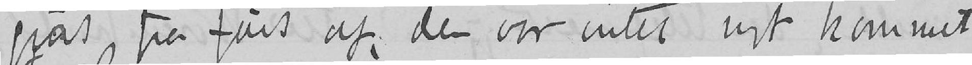gjort fra først af, der var intet nyt kommet
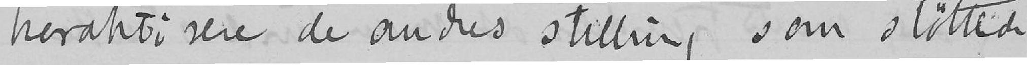karaktisere de andres stilling som støttede
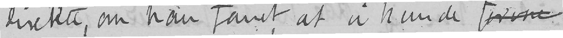direkte, om han fandt, at vi kunde forsvre
